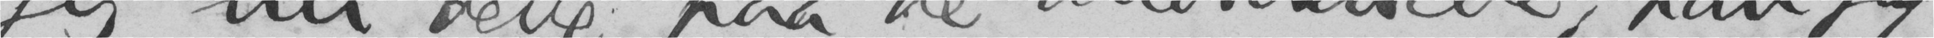jeg nu dette paa de allosexuelle; kan jeg
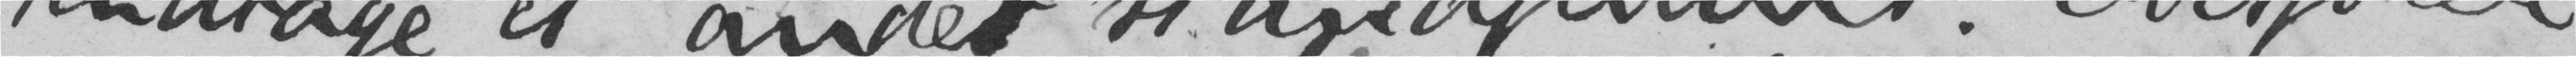indtage et andet standpunkt. Overfører
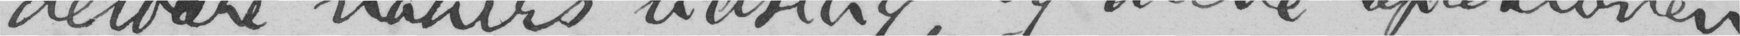delbare naturs udslag, og alene reflexionen,
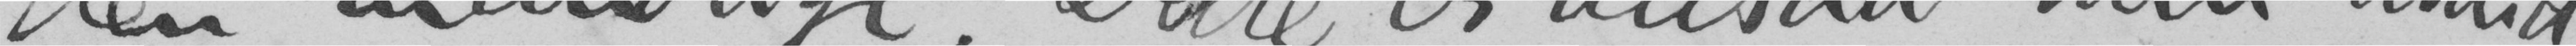den mandlige. Dette er altsaa min umid-
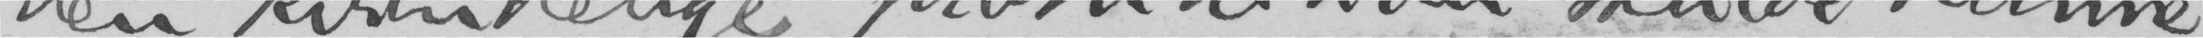den kvindelige prostitution skulde kunne
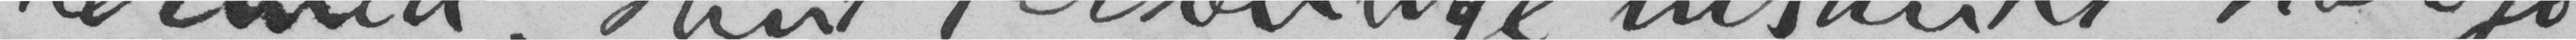hermed. Hint personlige instinkt har jo
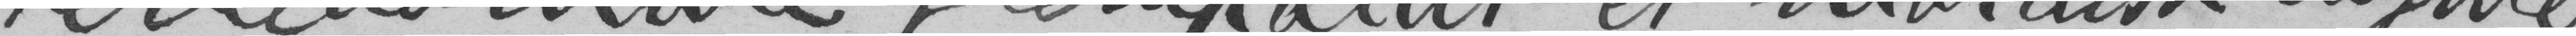herredømme fremkaldt et moralsk dogme,
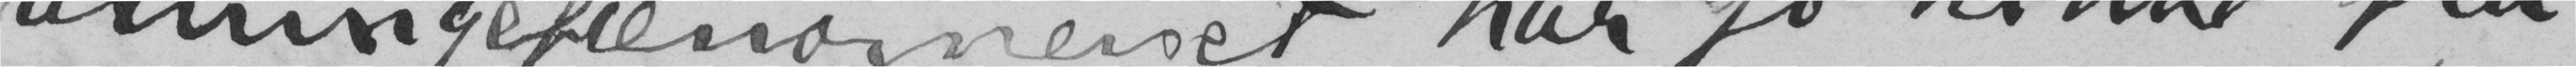urningefænomenet har jo hidtil fra
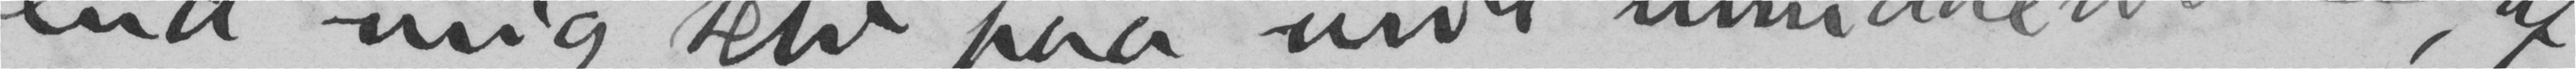end mig selv paa mit umiddelbare, af
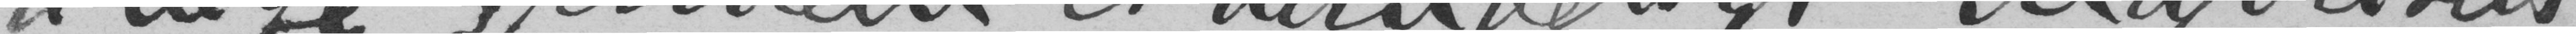tillige gjennem et aandeligt majoritets-
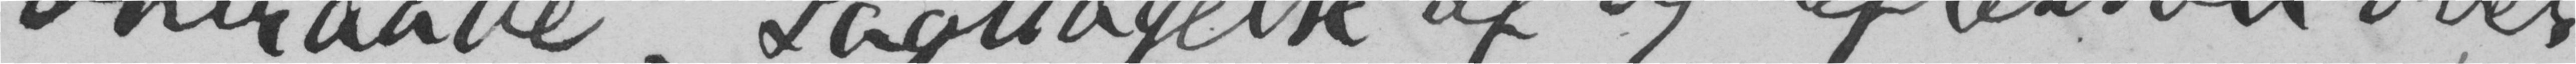omraade. Iagttagelse af og reflexion over
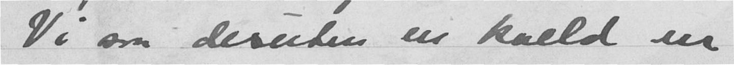Vi saa desuten en kveld en
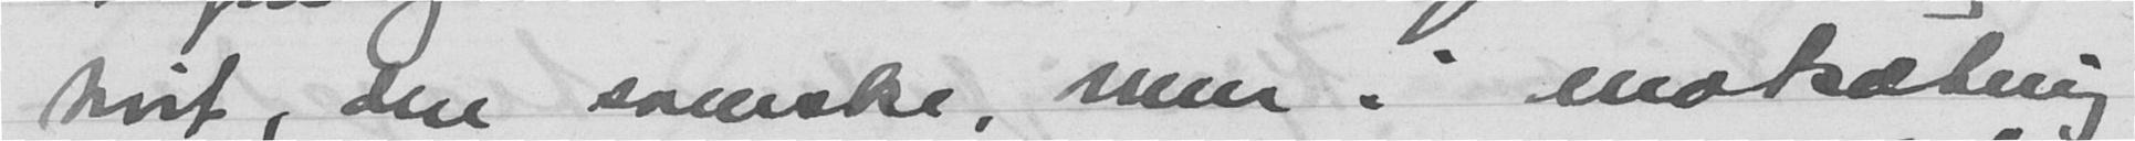hvit, den svenske, men i motsætning
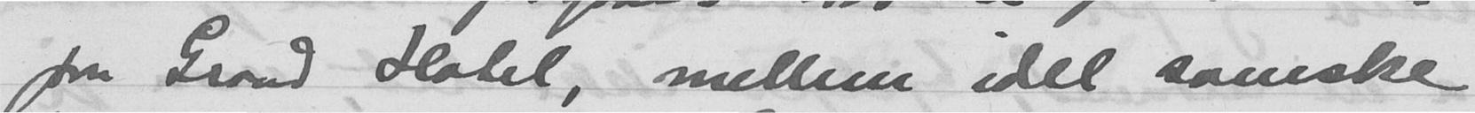paa Grand Hotel, mellem idel svenske
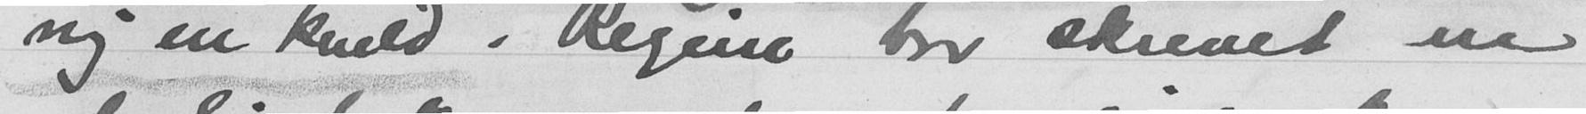mig en kveld. Regine har skrevet en
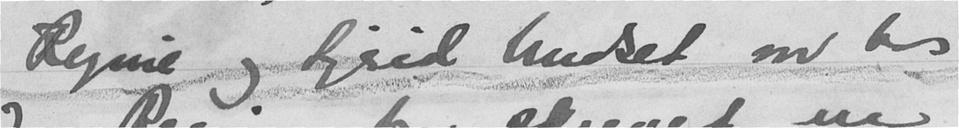Regine og Sigrid Undset var hos
